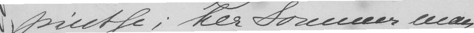pintse; her kommer man-
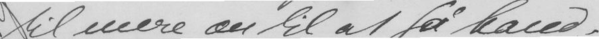til mere æn til at få hand-
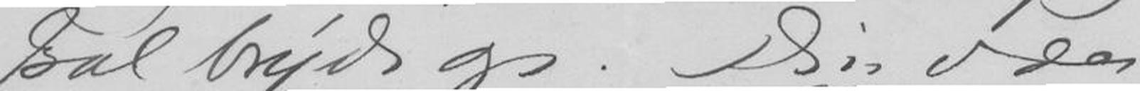skal bryde op. Din væn
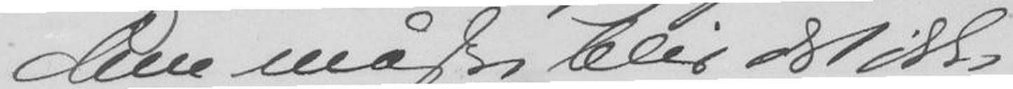Men måske blir det ikke
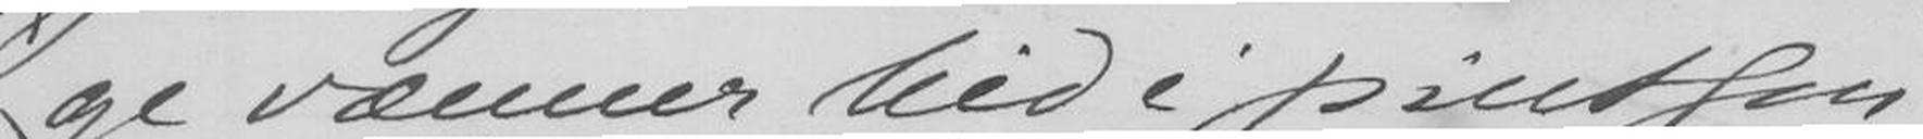ge vænner hid i pintsen
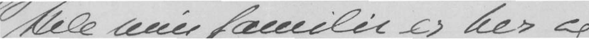Hele min familie er her og
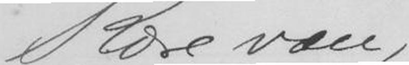Kære væn,
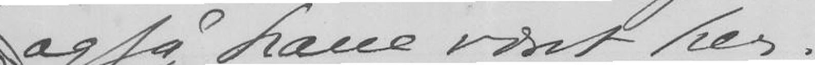også have været her! -
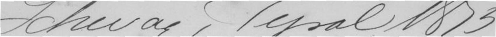Schwaz, Tyrol 1875
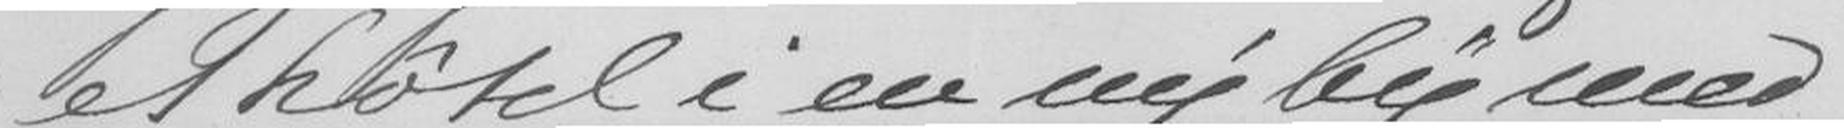et hôtel i en ny by med
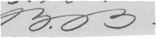B.B.
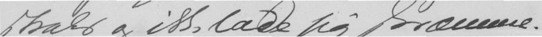straks og ikke lade sig skræmme.
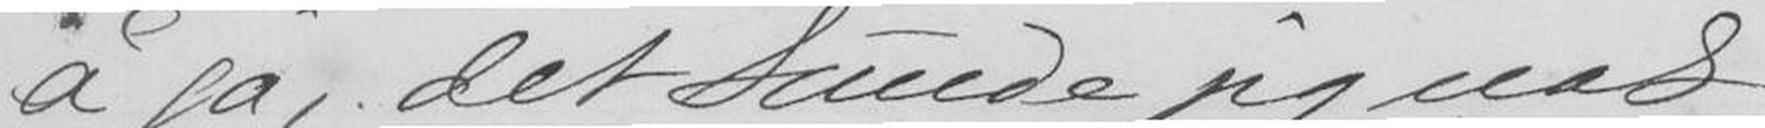Å ja, det kunde jeg nok
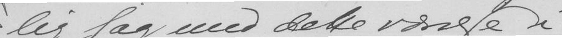lig sag med dette værelse i
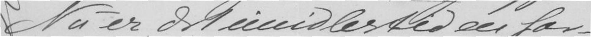Nu er det imidlertid en far-
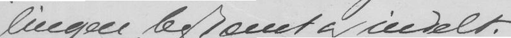lingen bestæmt og indelt.
